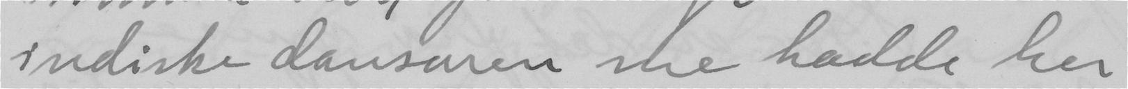indiske dansaren me hadde her
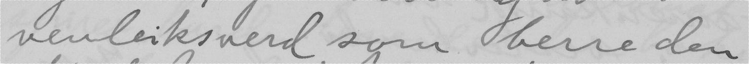venleiksverd som berre den
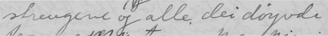strengene og alle dei döyvde
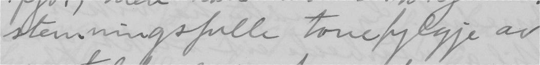stemningsfulle tonefylgje av
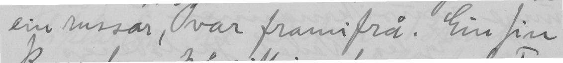ein russar, ovar framifrå. Ei fin
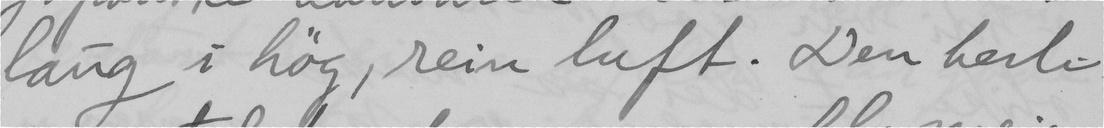laug i hög, rein luft. Den beste
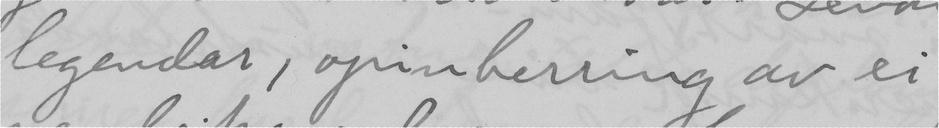legendar, opinberring av ei
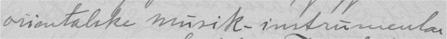orientalske musik-instrumentar
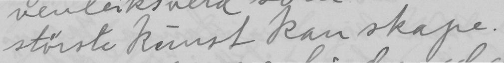störste kunst kan skape.
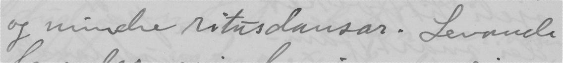og mindre ritusdansar. Levande
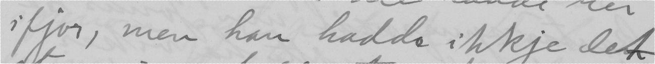ifjor, men han hadde ikkje det
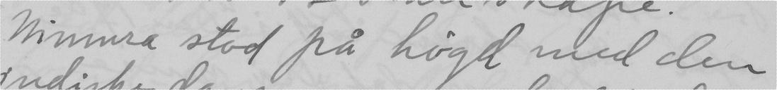Nimmra stod på högd med den
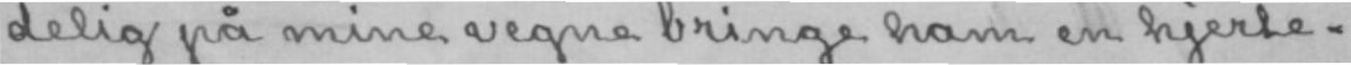delig på mine vegne bringe ham en hjerte-
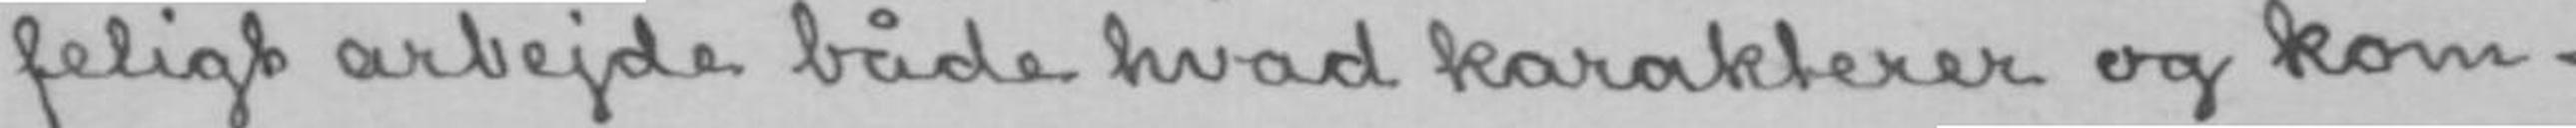feligt arbejde både hvad karakterer og kom-
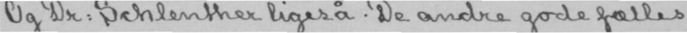Og Dr: Schlenther ligeså. De andre gode fælles
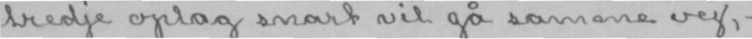tredje oplag snart vil gå samme vej,-
